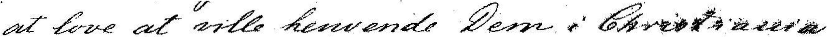at love at ville henvende Dem i Cristiania
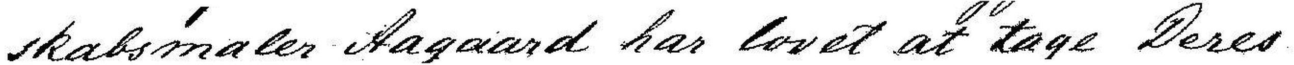skabsmaler Aagaard har lovet at tage Deres
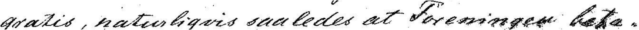gratis, naturligvis saaledes at Foreningen beta-
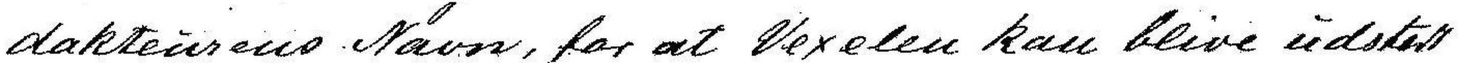dakteurens Navn, for at Vexelen kan blive udstedt
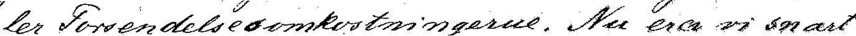ler Forsendelsesomkostningerne. Nu ere vi snart
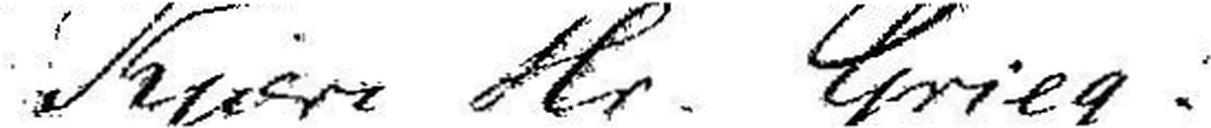Kjære Hr. Grieg!
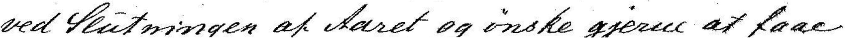ved Slutningen af Aaret og ønske gjerne at faae
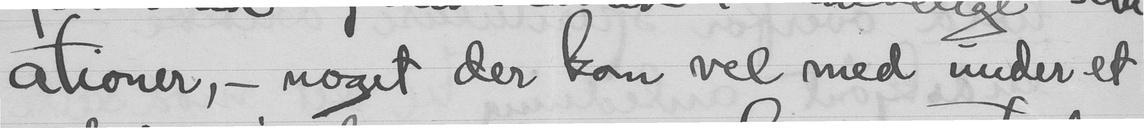ationer, - noget der kan vel med under et
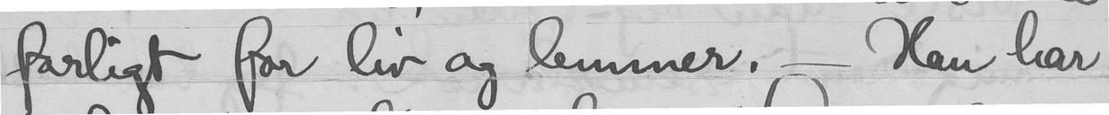farligt for liv og lemmer. - Han har
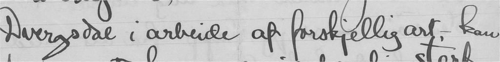Dvergsdal i arbeide af forskjellig art, - kan
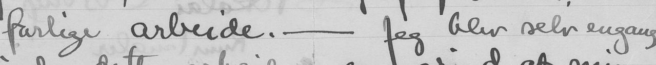farlige arbeide. - Jeg blev selv engang
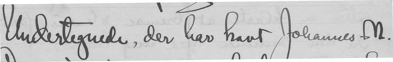Undertegnede, der har havt Johannes N.
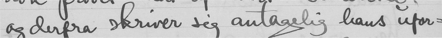og derfra skriver sig antagelig hans ufor-
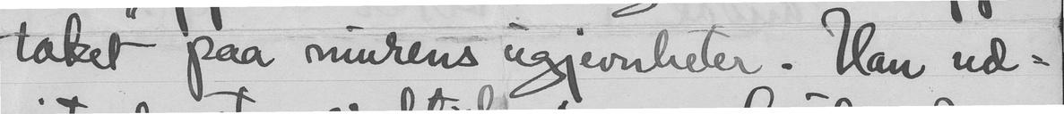taket paa murens ugjevnheter. Han ud-
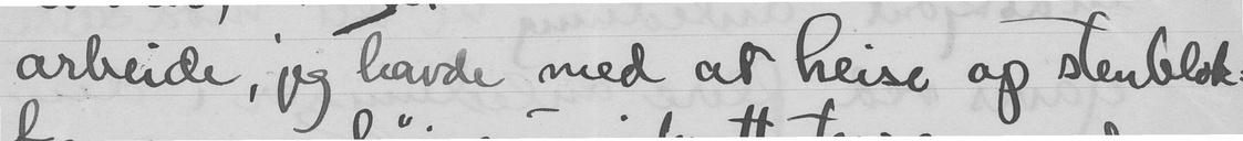arbeide, jeg havde med at heise op stenblok-
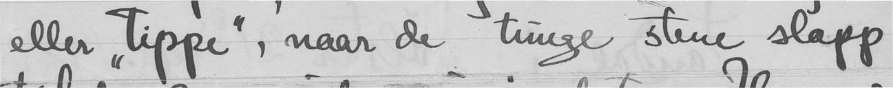eller "tippe", naar de tunge stene slapp
